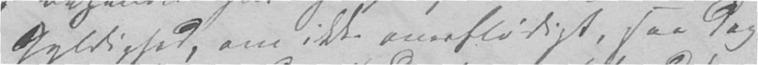Gyldighed, om ikke overflødigt, saa dog
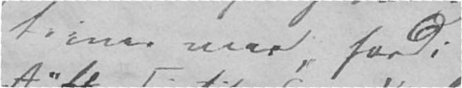triner maa, fordi
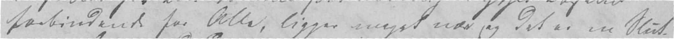forbindende for Alle, ligger meget nær og det er en Slut-
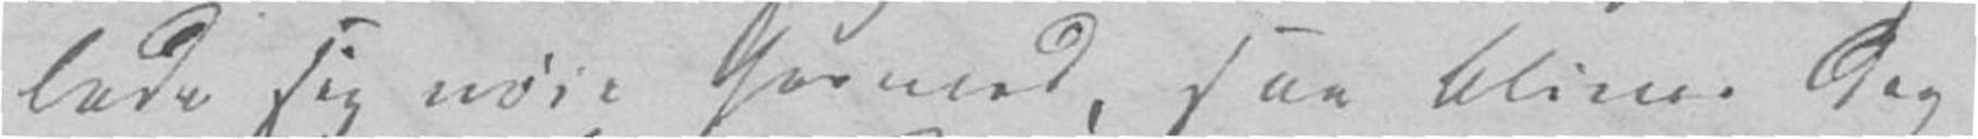lade sig nøie hermed, saa bliver dog
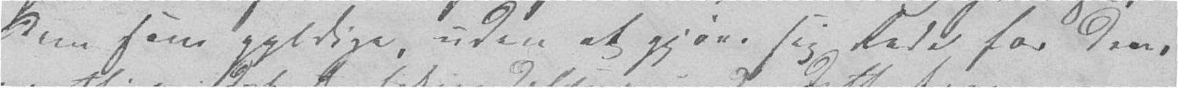dem som gyldige, uden at gjøre sig Rede for deres
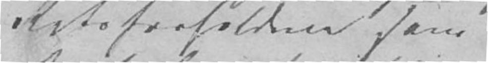Retsforholdene som
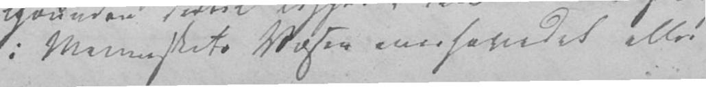i Menneskets Væsen overhovedet eller
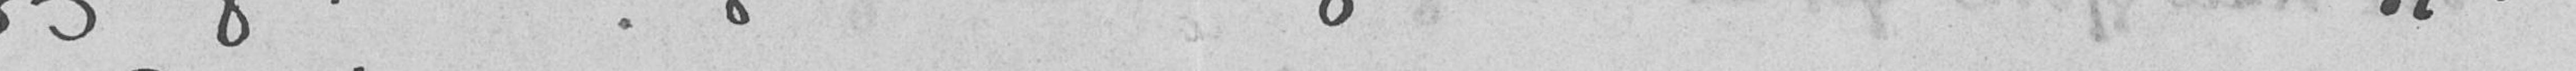gig af støbningsomkostningerne. Kan du ikke gjøre
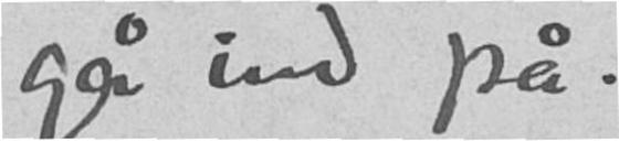gå ind på.
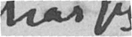har jeg
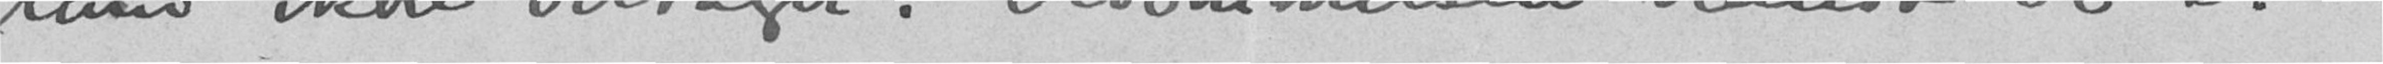land ikke deltager i bedømmelsen blandt de 3."
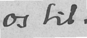os til.
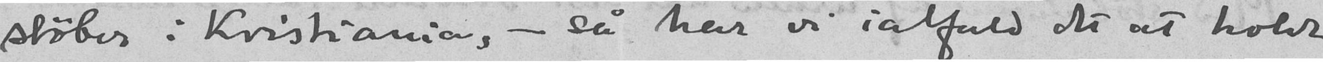støber i Kristiania, - så har vi ialfald det at holde
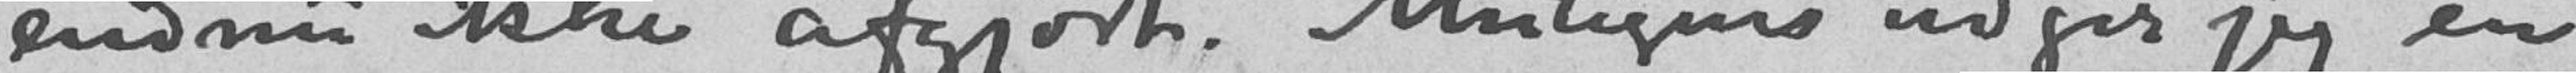endnu ikke afgjort. Muligens udgir jeg en
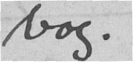bog.
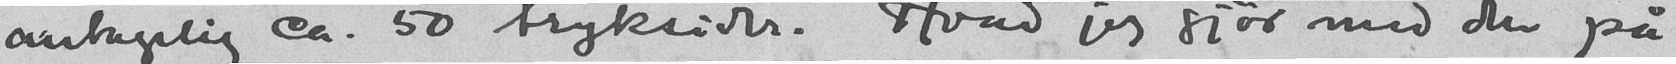antagelig ca. 50 tryksider. Hvad jeg gjør med den på
